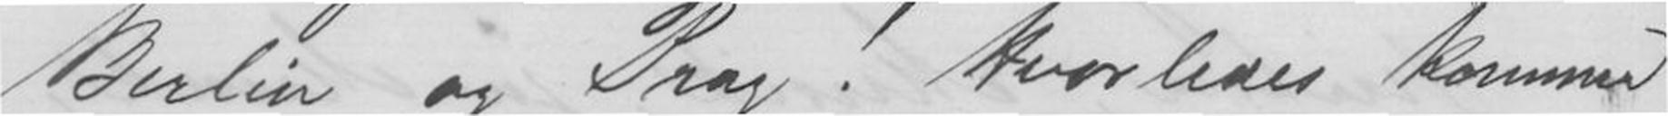Berlin og Prag! Hvorledes kommer
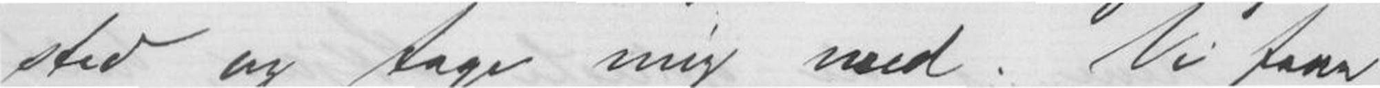sted og tage mig med. Vi faar
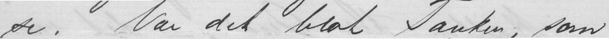se. Var det blot Tanken, som
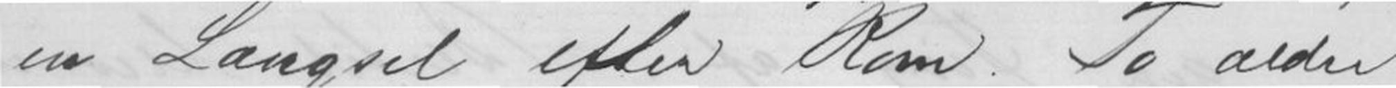en Længsel efter Rom. To ældre
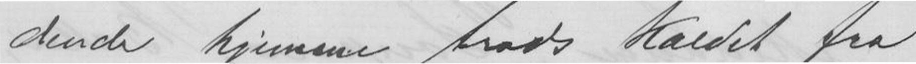dende hjemme trods Kaldet fra
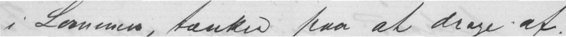i Lommen, tænker paa at drage af-
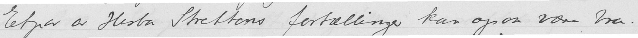Etpar av Hesba Strettons fortællinger kan ogsaa være bra.
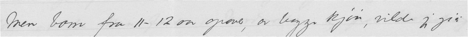Men barn fra 11-12 aar opover, av begge kjøn, vilde jeg gi:
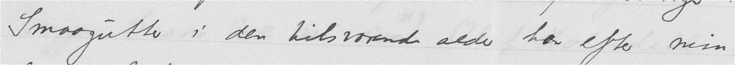Smaagutter i den tilsvarende alder har efter min
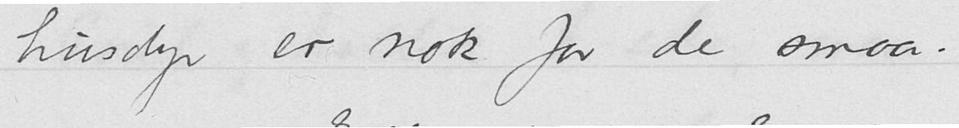husdyr er nok for de smaa.
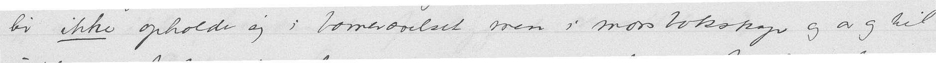liv ikke opholde sig i barneværelset, men i mors bokskap og av og til
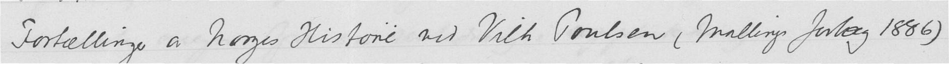Fortællinger av Norges Historie ved Vilh Poulsen (Mallings forlag 1886)
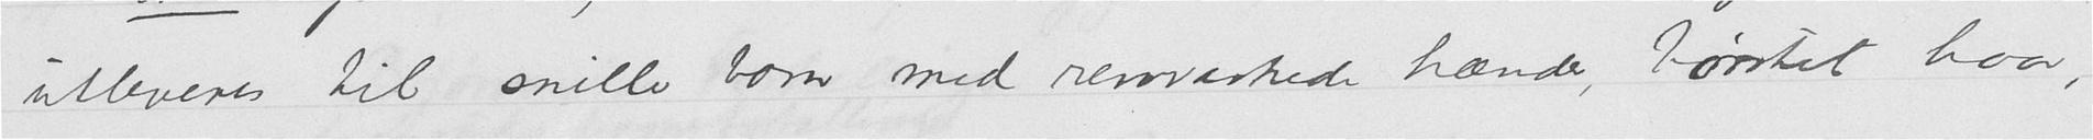utleveres til snille barn med renvaskede hænder, børstet haar,
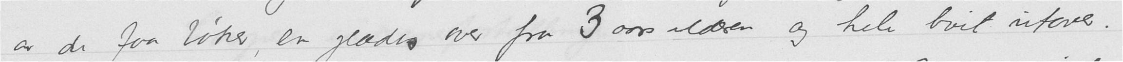av de faa bøker, en glædes over fra 3 aars alderen og hele livet utover.
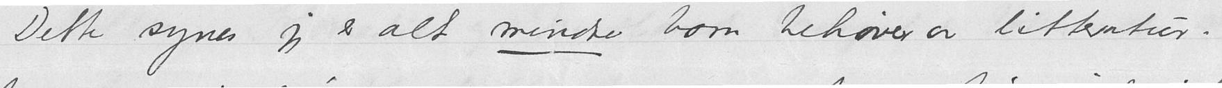Dette synes jeg er alt mindre barn behøver av litteratur.
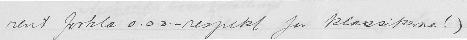rent forklæ o.s v. - respekt for klassikerne!)
